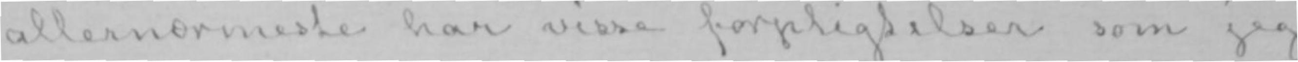allernærmeste har visse forpligtelser som jeg
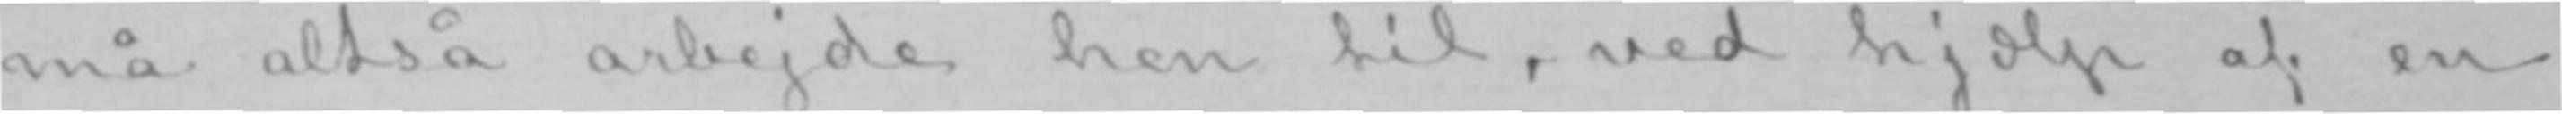må altså arbejde hen til, ved hjælp af en
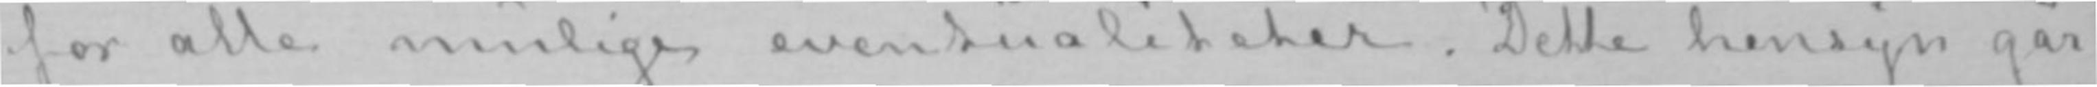for alle mulige eventualiteter. Dette hensyn går
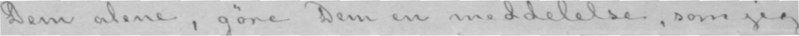Dem alene, gøre Dem en meddelelse, som jeg
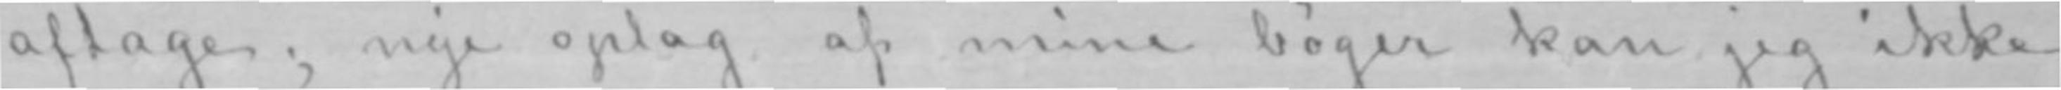aftage; nye oplag af mine bøger kan jeg ikke
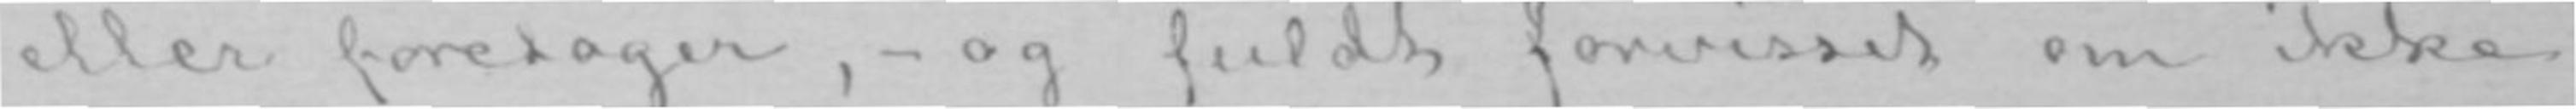eller foretager,- og fuldt forvisset om ikke
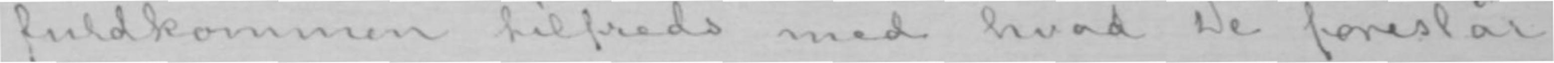fuldkommen tilfreds med hvad De foreslår
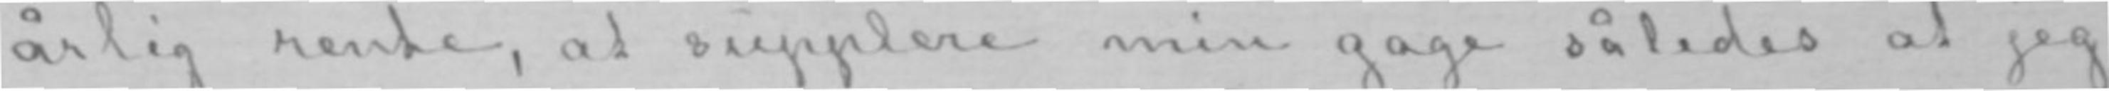årlig rente, at supplere min gage således at jeg
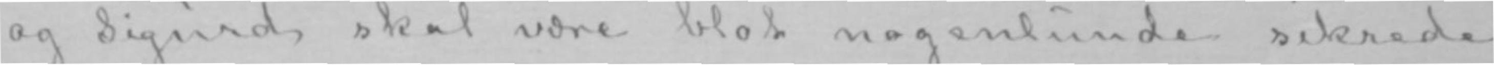og Sigurd skal være blot nogenlunde sikrede
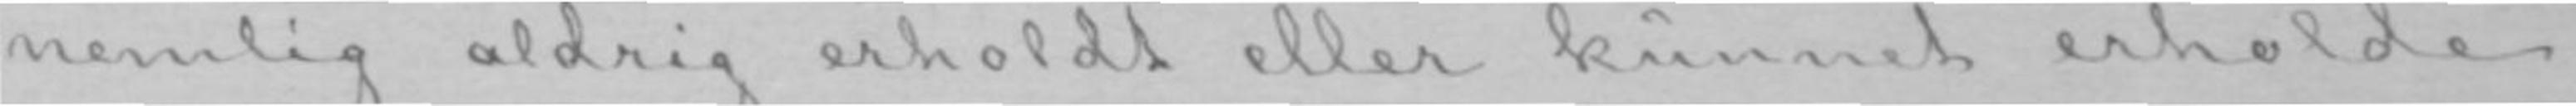nemlig aldrig erholdt eller kunnet erholde
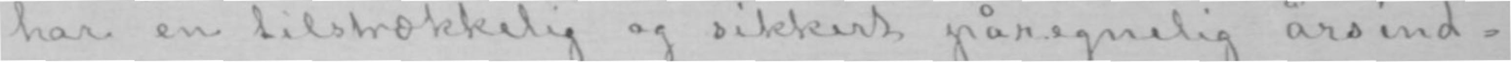har en tilstrækkelig og sikkert påregnelig årsind-
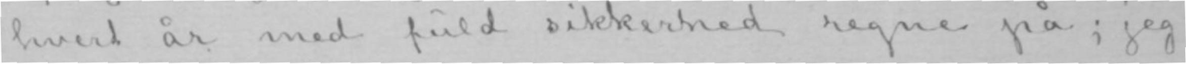hvert år med fuld sikkerhed regne på; jeg
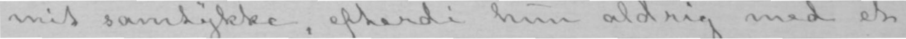mit samtykke, efterdi hun aldrig med et
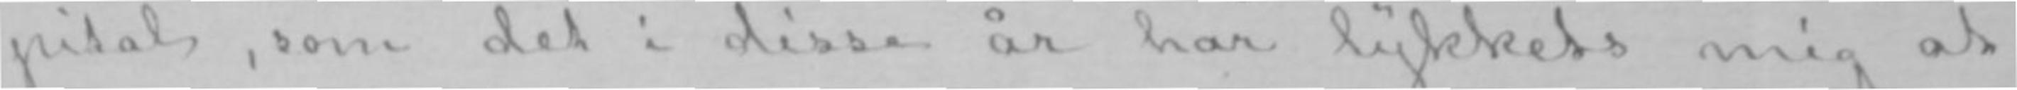pital, som det i disse år har lykkets mig at
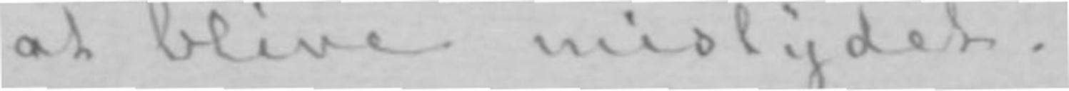at blive mistydet.
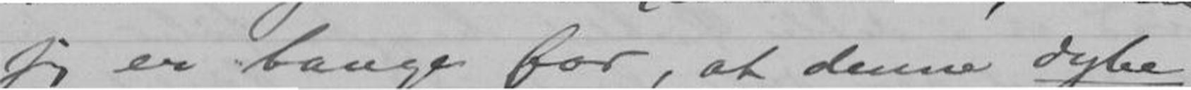jeg er bange for, at denne dybe
